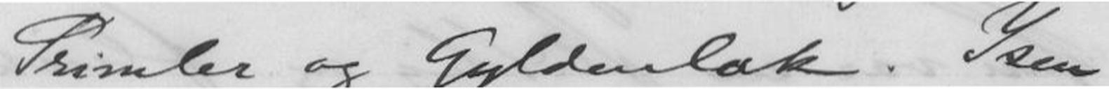Primler og Gyldenlak. Isen
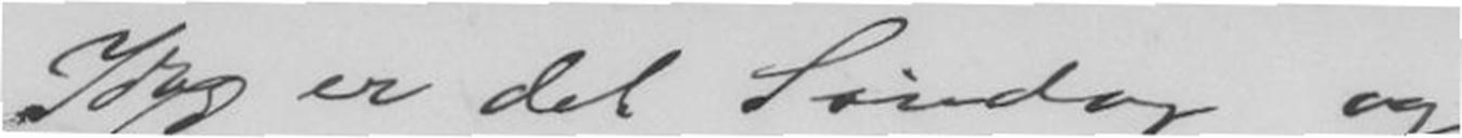Idag er det Søndag og
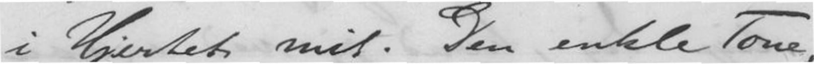i Hjertet mit. Den enkle Tone,
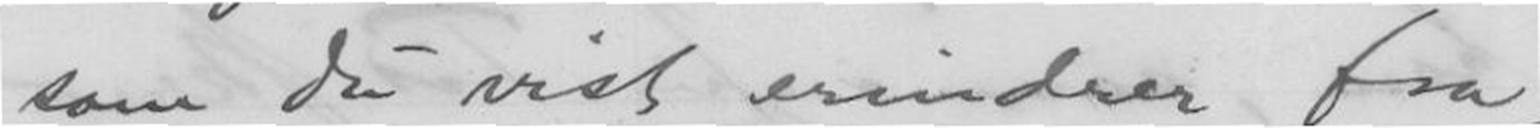som du vist erindrer fra
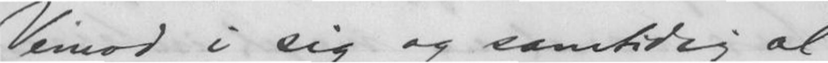Vemod i sig og samtidig al
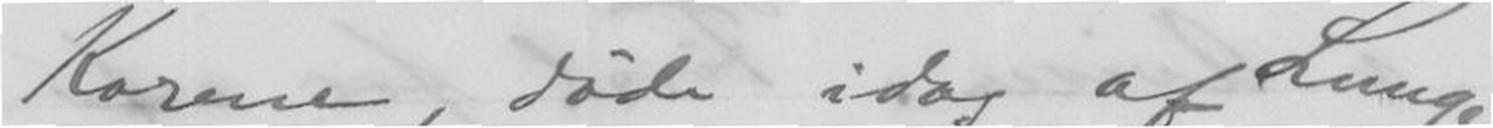Korene, døde idag af Lunge-
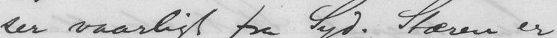ser vaarligt fra Syd. Stæren er
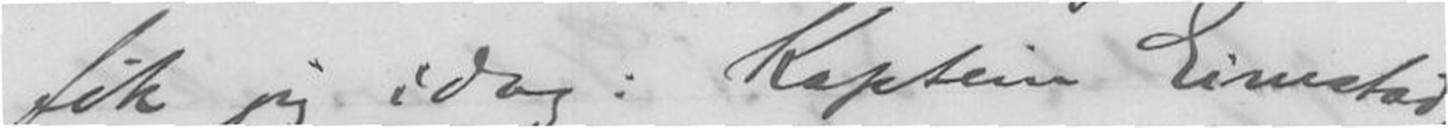fik jeg idag. Kaptein Eimstad,
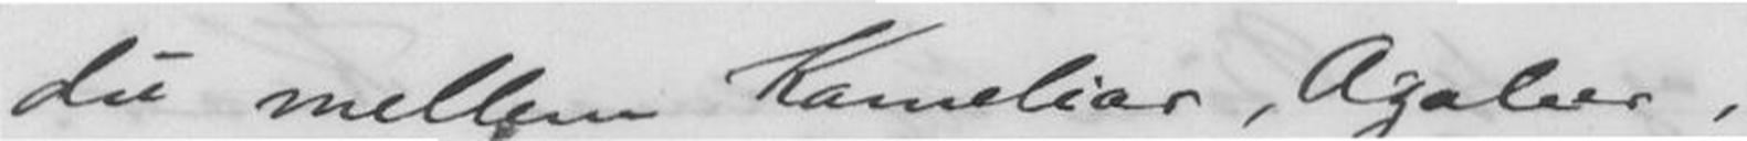du mellem Kameliar, Azalaer,
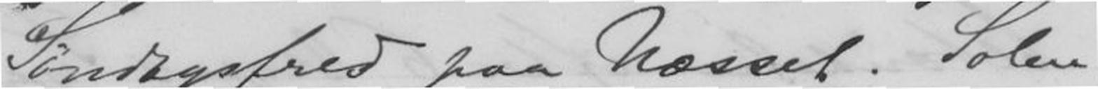Søndagsfred paa Næsset. Solen
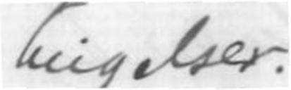tingelser.
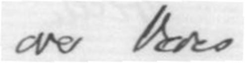over vores
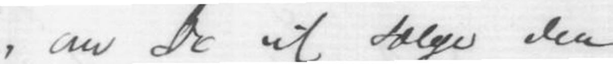, om De vil sælge den
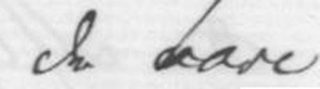da have
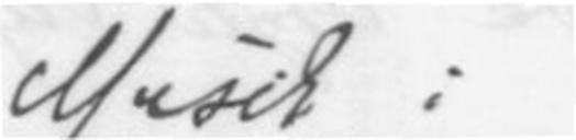Musik i
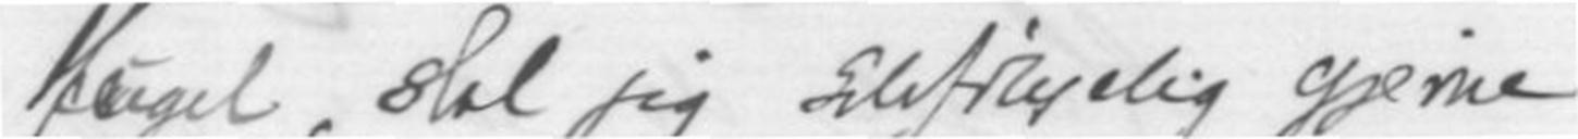Heugel, skal jeg selvfølgelig gjerne
